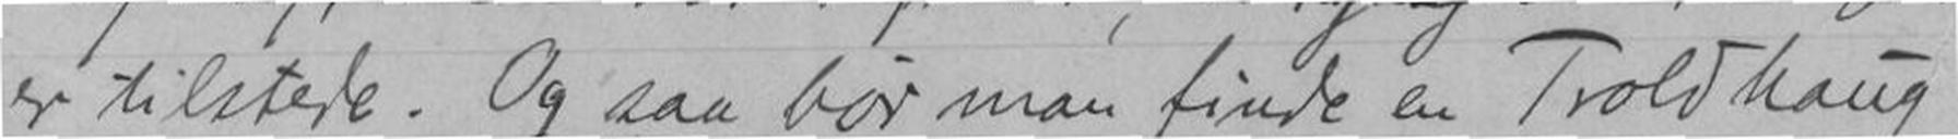er tilstedet. Og saa bør man finde en Troldhaug
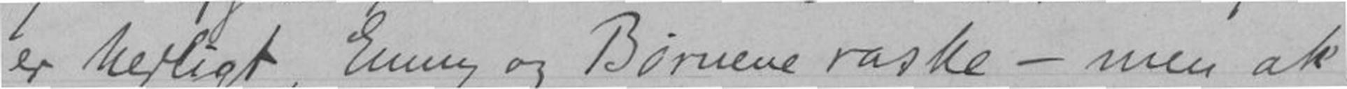er herligt, Emmy og Børnene raske - men ak,
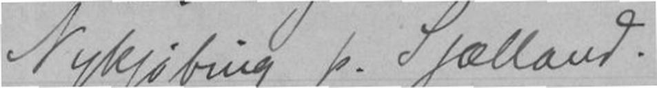Nykjøbing p. Sjælland
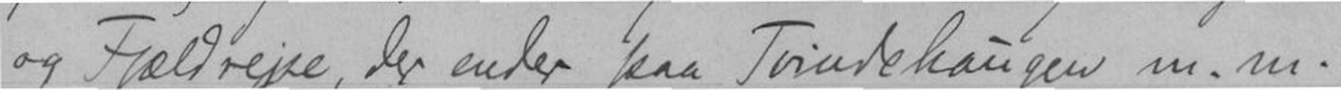og Fjælderejse, der ender paa Tvindehaugen m. m.
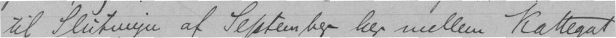til Slutningen at September her mellem Kattegat
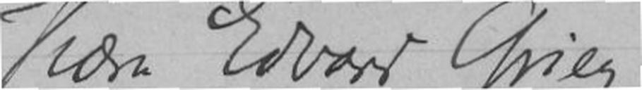Kære Edvard Grieg
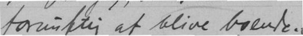fornuftig at blive boende.
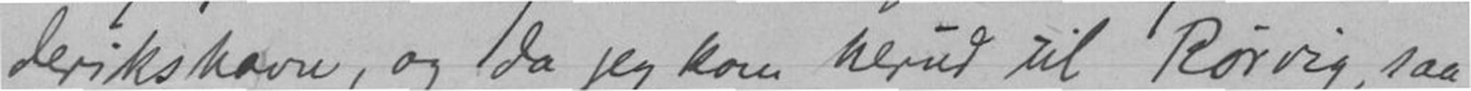drikshavn, og da jeg kom herud til Rørvig, saa
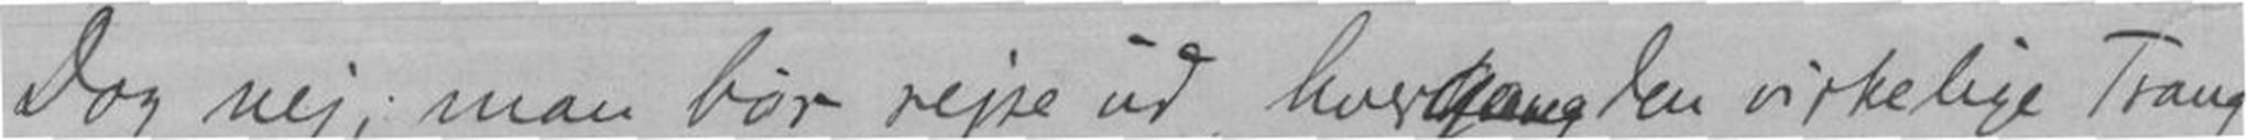Dog nej, man bør rejse ud, hver gang den virkelige Trang
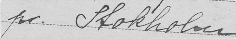pr. Stokholm
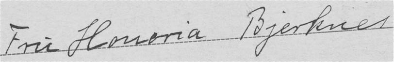Fru Honoria Bjerknes
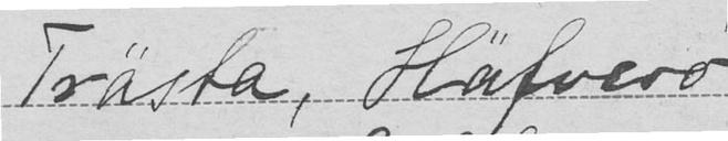Trästa, Häfvero
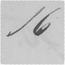16
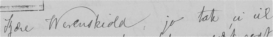Kjære Werenskiold, jo tak, vi vil
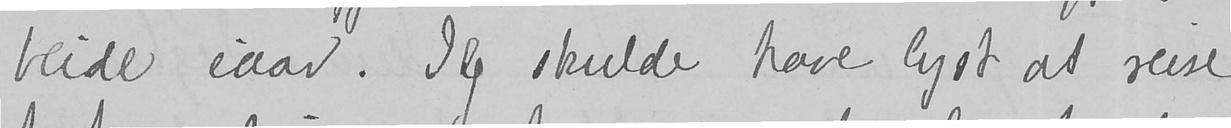beide iaar. Jeg skulde have lyst at reise
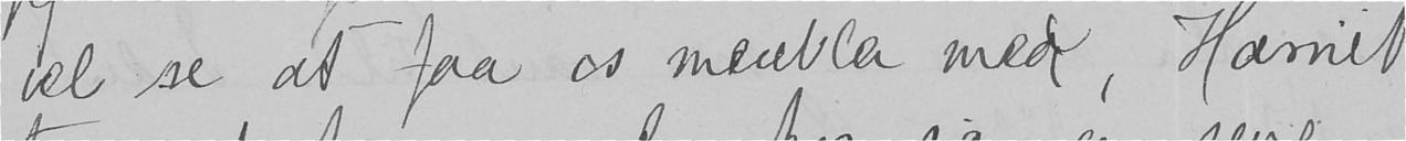vel se at faa os meubler med, Harriet
